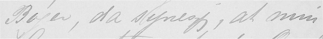Bøger, da synes jeg, at min
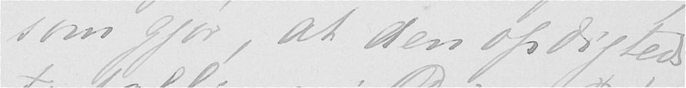som gjør, at den opdigtede
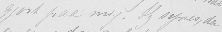gjørt paa mig. Jeg synes, den
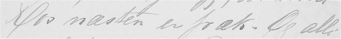Ros næsten er fræk. Og alli-
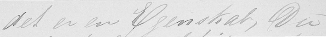det er en Egenskab, Du
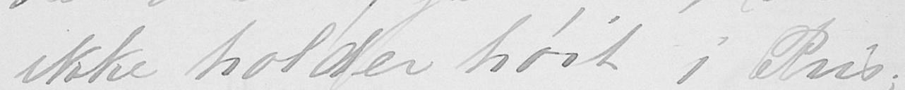ikke holder høit i Pris;
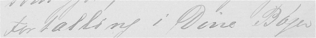Fortælling i Dine Bøger
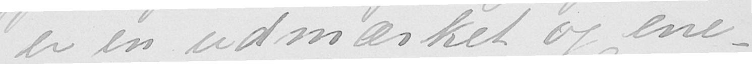er en udmærket og ene-
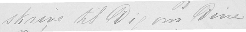skrive til Dig om Dine
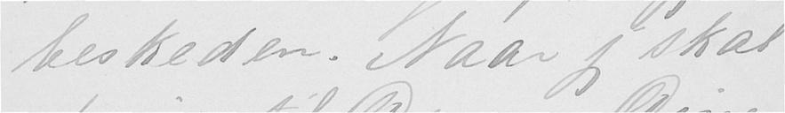beskeden. Naar jeg skal
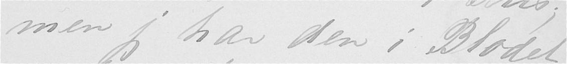men jeg har den i Blodet,
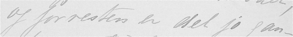og forresten er det jo gan-
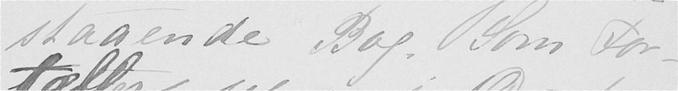staaende Bog. Som For-
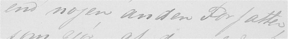end nogen anden Forfatter,
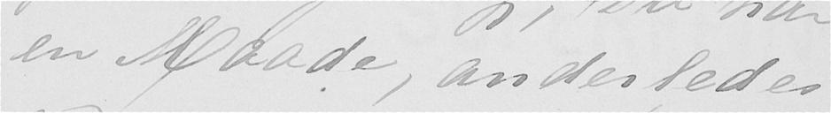en Maade, anderledes
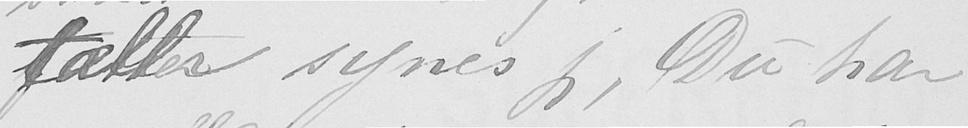tæller synes jeg, Du har
